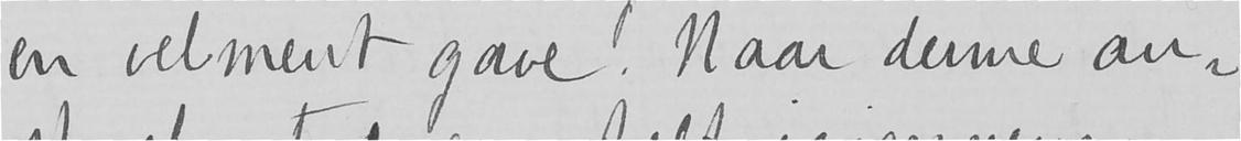en velment gave. Naar denne an-
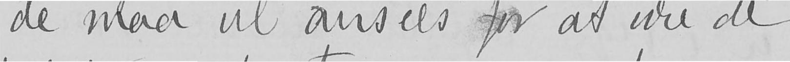de maa vel ansees for at være de
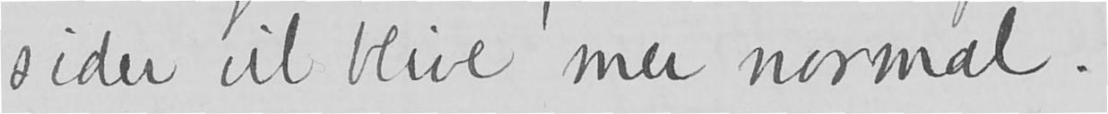sider vil blive mer normal.
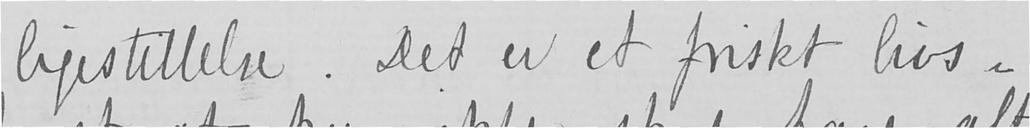ligestillelse. Det er et friskt livs-
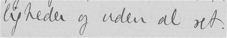ligheder og uden al ret.
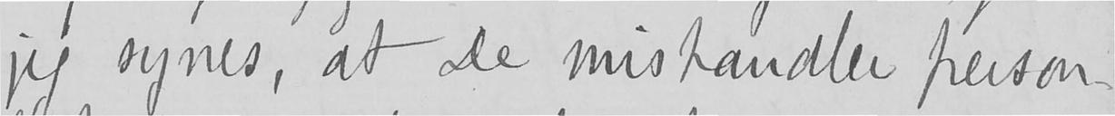jeg synes, at De mishandler person-
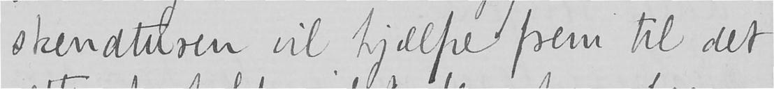skenaturen vil hjælpe frem til det
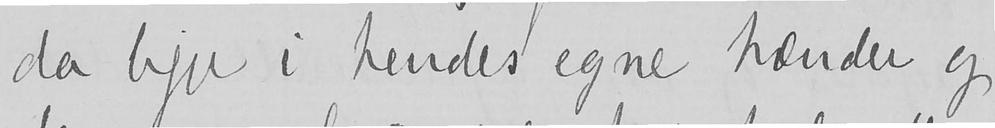da ligge i hendes egne hænder og
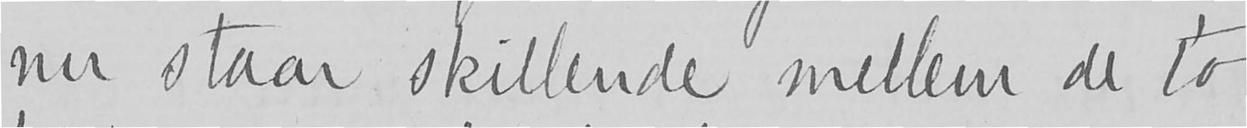nu staar skillende mellem de to
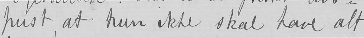pust, at hun ikke skal have alt
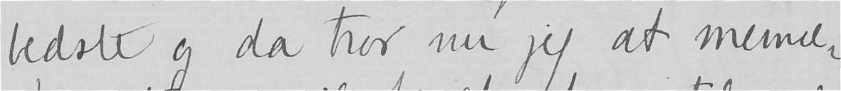bedste og da tror nu jeg at menne-
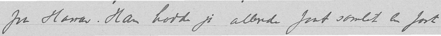fra Hamar. Han hadde jo allerede faat samlet en fast
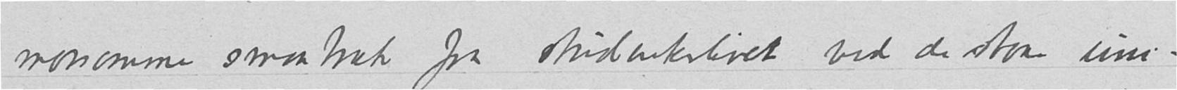morsomme smaatræk fra studenterlivet ved de store uni-
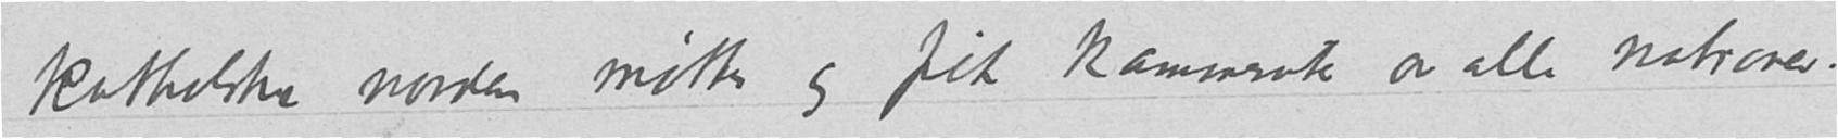katholske norden møttes og fik kammerater av alle nationer.
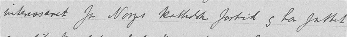interesseret for Norges katholske fortid og har fattet
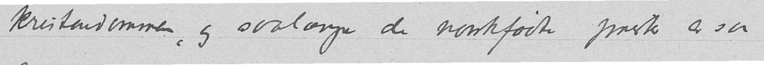kristendommen, og saalænge de norskfødte præster er saa
